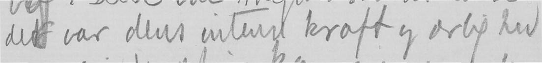det var dens intense kraft og ærlighed
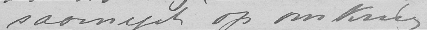saameget op omkring
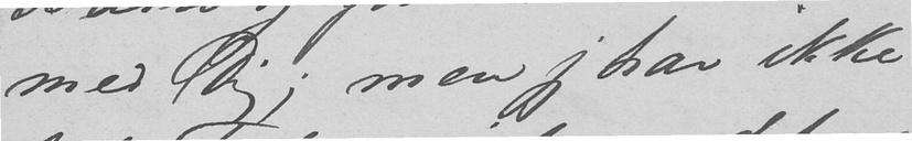med Dig; men jeg har ikke
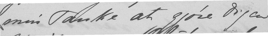min Tanke at gjøre Dig en
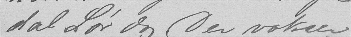dal Lørdag. Der vokser
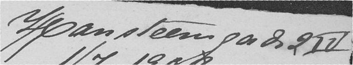Hansteens gade 2 IV
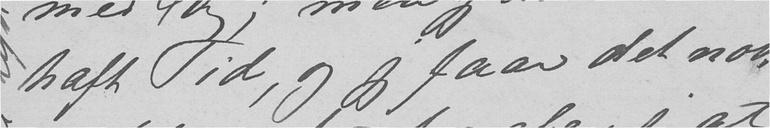haft Tid, og jeg faar det nok
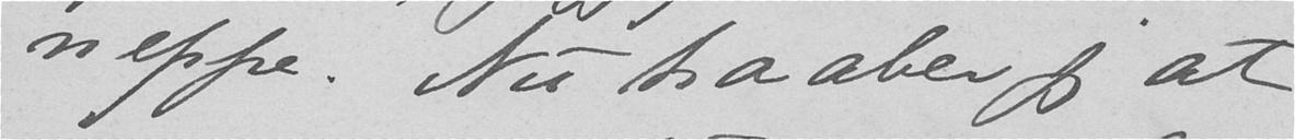neppe. Nu haaber jeg at
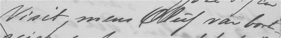Visit mens Oluf var bort-
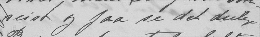reist og faa se det deilige
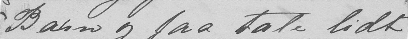Barn og faa tale lidt
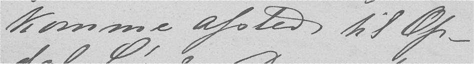komme afstede til Op-
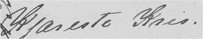Kjæreste Kris.
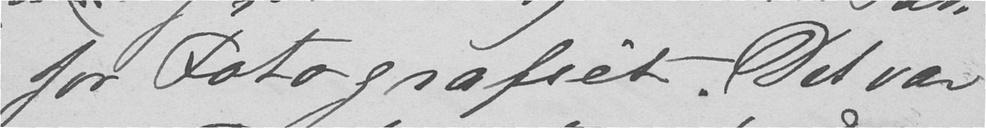for Fotografiet. Det var
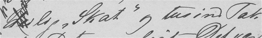deilig "Skat" og tusind Tak-
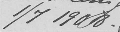1/7 1908
